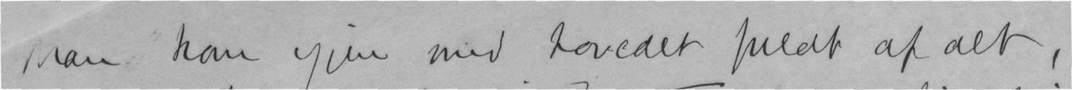man kan igjen med hovedet fuldt af alt,
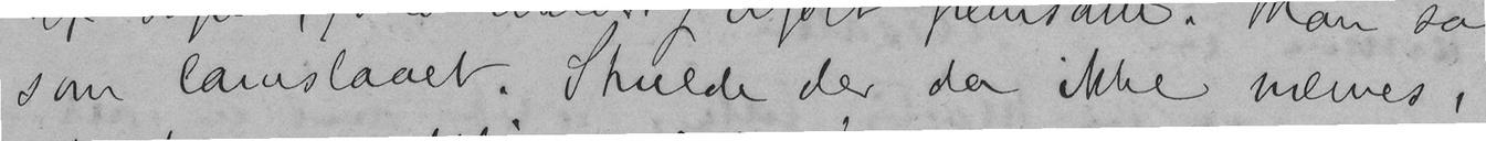som lamslaaet. Skulde der da ikke nævnes,
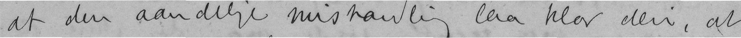at den aandelige mishandling laa klar deri, at
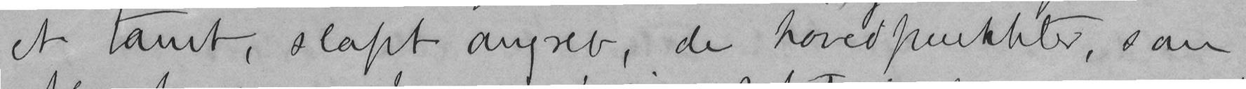et tamt, slapt angreb, de hovedpunkter, som
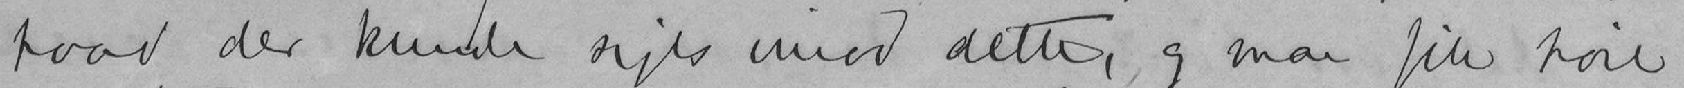hvad der kunde siges imod dette, og man fik høre
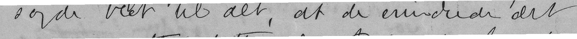sagde blot til alt, at de erindrede det
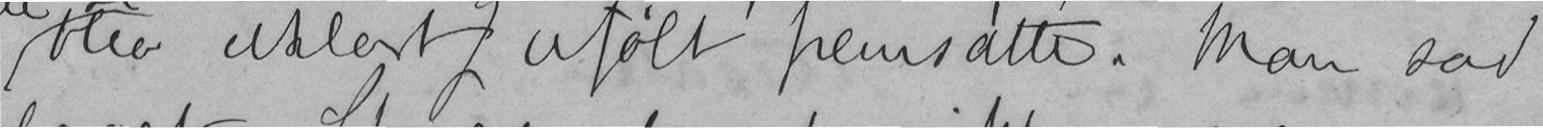blev erklært f ufølt fremsatte. Man sad
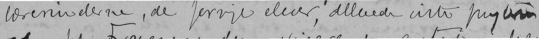lærerinderne, de forrige elever, allerede viste frugterne
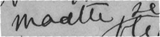maatte se
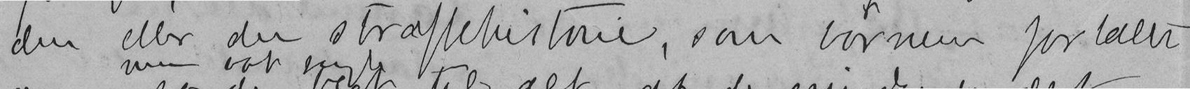den eller den straffehistorie, som børnene fortalte
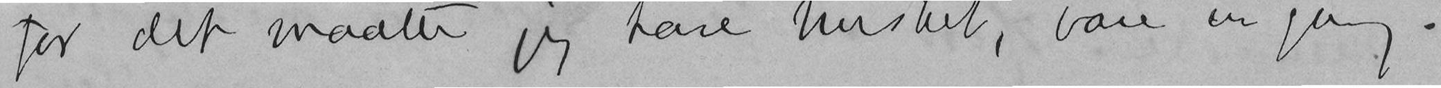for det maatte jeg have husket, bare en gang.
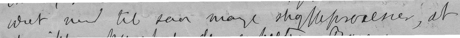været med til saa mange skogtteprocesser, at
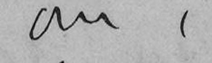om,
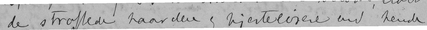de straffede haardere og hjerteløsere end hende
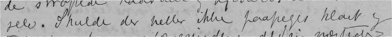selv. Skulde der heller ikke paapeges klart og
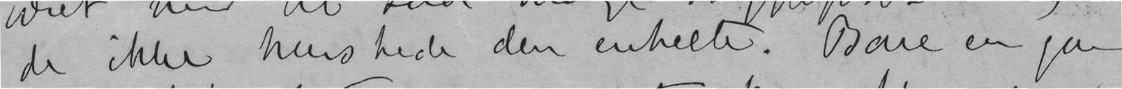de ikke huskede den enkelte. Bare en gang
